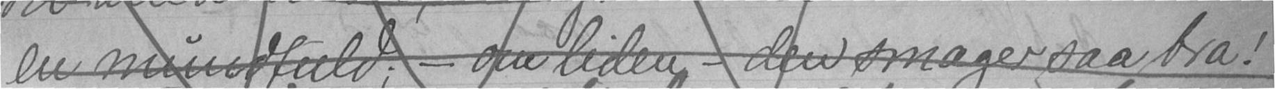en mundfuld; - om liden - den smager saa bra!
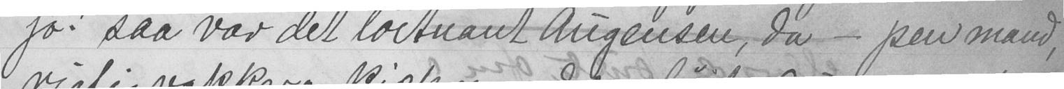jo! saa var det løitnant Augensen, da - pen mand,
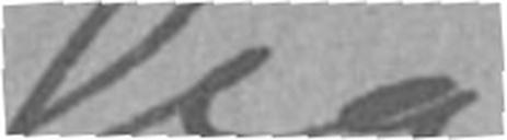ha
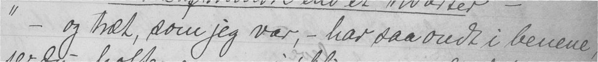" - og træt, som jeg var, - har saa ondt i benene,
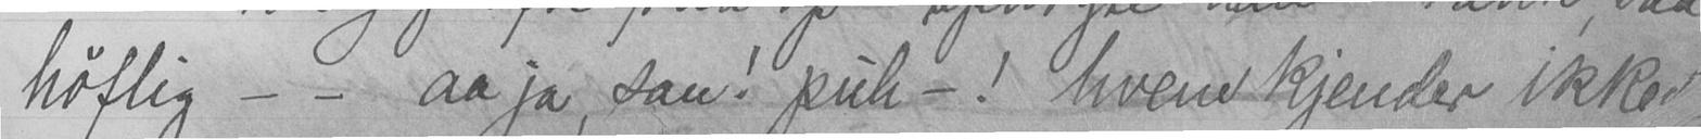høflig - - aa ja, san! puh - ! hvem kjender ikke
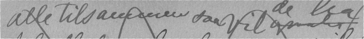alle tilsammen saa vil gaader
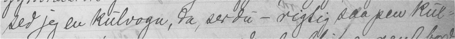sed jeg en kulvogn, da, ser du - rigtig saa pen kul-
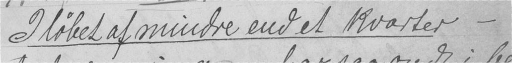I løbet af mindre end et kvarter -
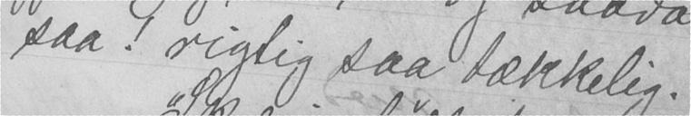saa! rigtig saa tækkelig.
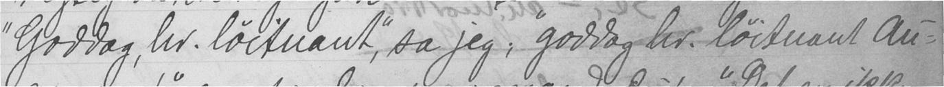"Goddag, hr. løitnant," sa jeg; goddag hr. løitnant Au-
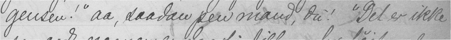gensen!" aa, saadan pen mand, du! "Det er ikke
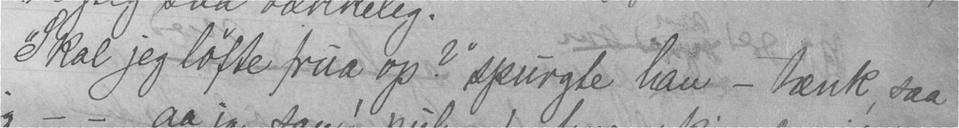"Skal jeg løfte frua op?" spurgte han - tænk, saa
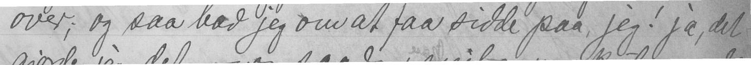over; og saa bad jeg om at faa sidde paa, jeg! ja, det
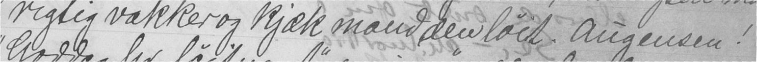rigtig vakker og kjæk mand den løit. Augensen!
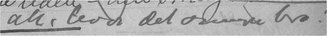alt, leva, det samme bro!
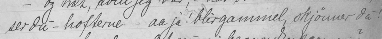ser du - hofterne - aa ja! blir gammel, skjønner du!
